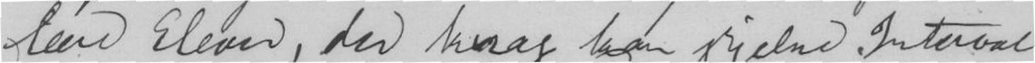lære Elever, der knap kan skjelne Interval-
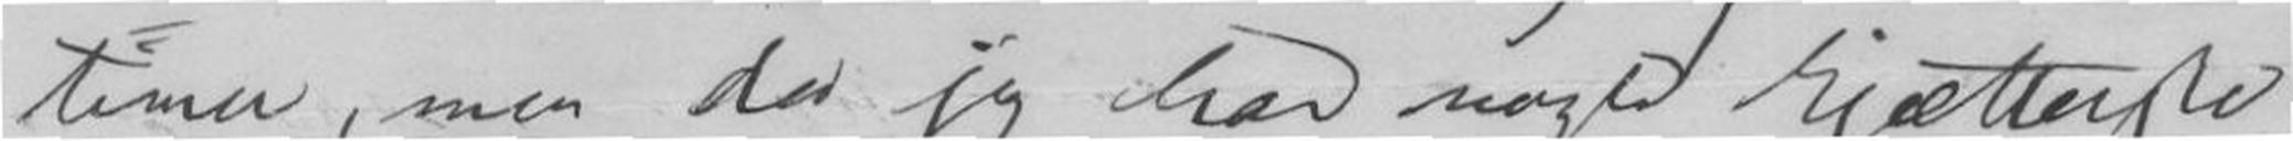timer, men da jeg har nogle kjætterske
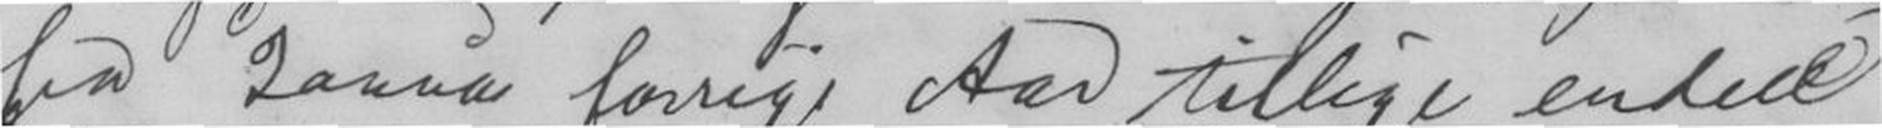fra Januar forrige Aar tillige endeel
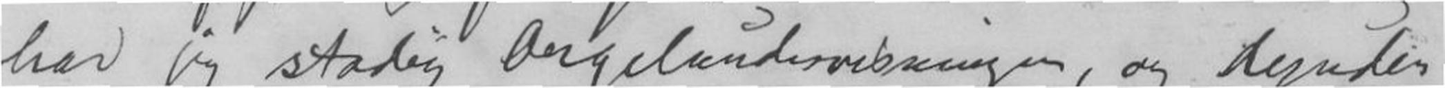har jeg stadig Orgelundervisningen, og desuden
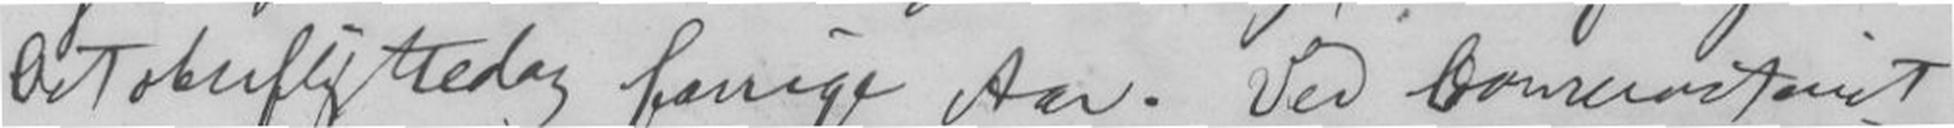Octoberflyttedag forrige Aar. Ved Concervatoriet
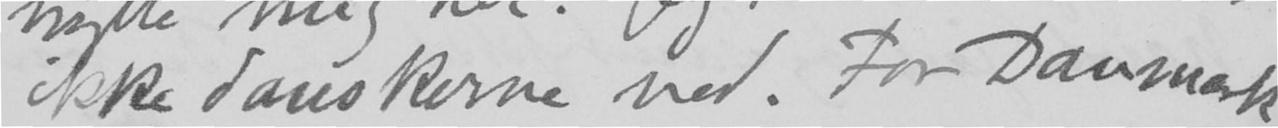ikke danskerne ved. For Danmark
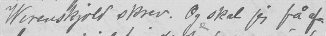Werenskjold skrev. Og skal jeg få af-
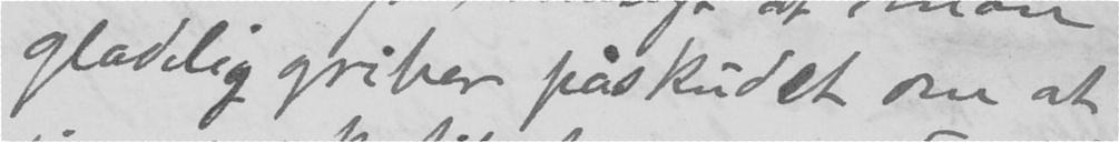gladelig griber påskudet om at
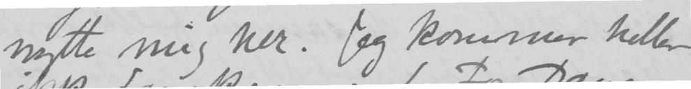nytte mig her. Jeg kommer heller
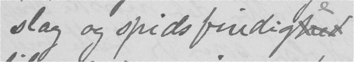slag og spidsfindighed
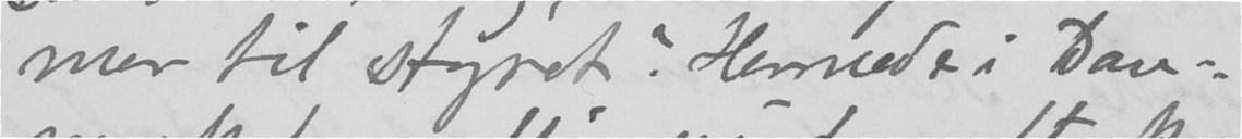mer til Styret? Hernede i Dan-
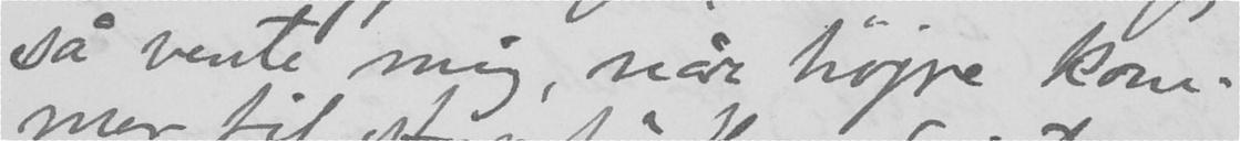så vente mig, når højre kom-
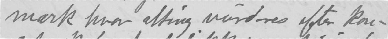mark hvor alting vurderes efter kon-
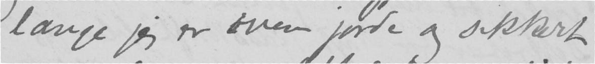længe jeg er oven jorde og sikkert
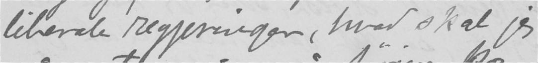liberale regjeringer, hvad skal jeg
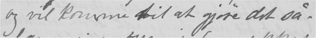og vil komme til at gjøre det så-
